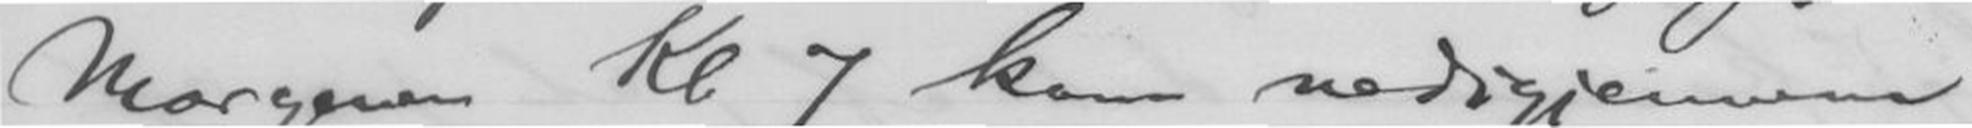Morgen Kl 7 kom nedigjennem
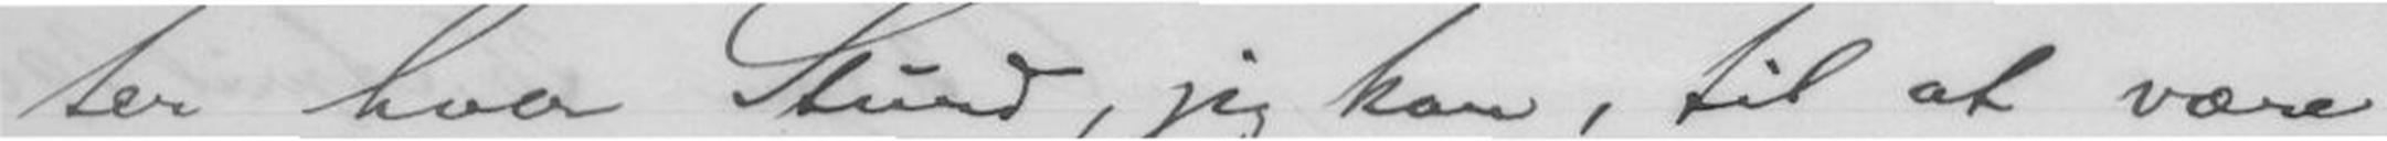ter hver Stund, jeg kan, til at være
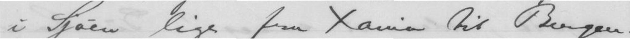i Sjøen lige fra Xania til Bergen.
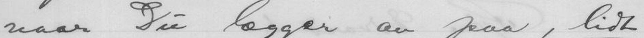naar Du lægger an paa, lidt
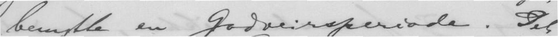benytte en Godveirsperiode. Det
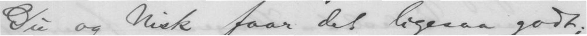Du og Nisk faar det ligesaa godt;
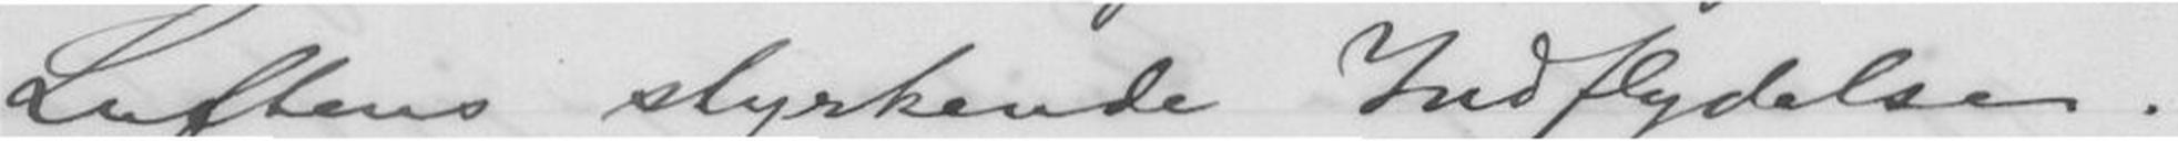Luftens styrkende Indflydelse.
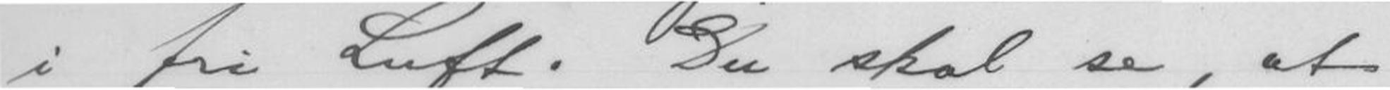i fri Luft. Du skal se, at
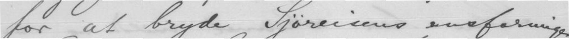for at bryde Sjøreisens ensformige
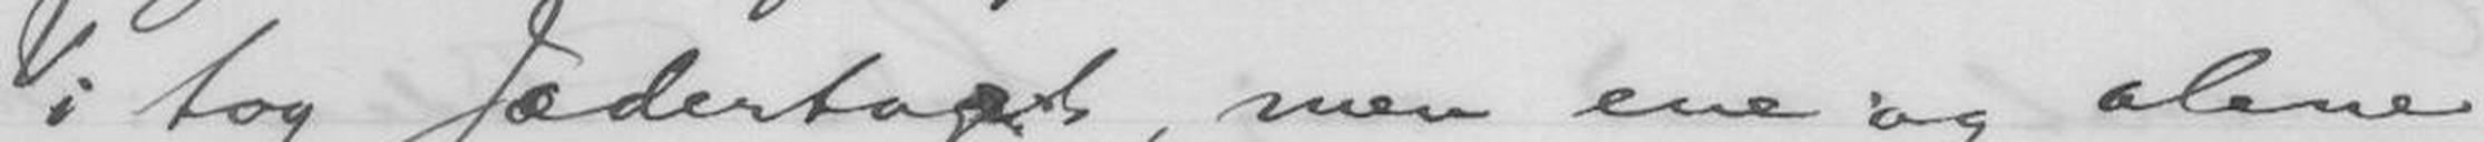Vi tog Jædertoget, men ene og alene
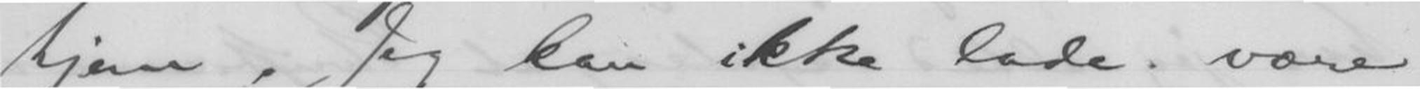hjem. Jeg kan ikke lade være
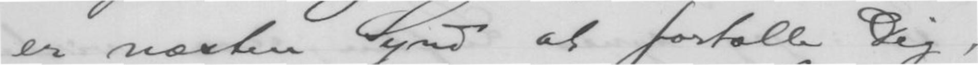er næsten Synd at fortælle Dig,
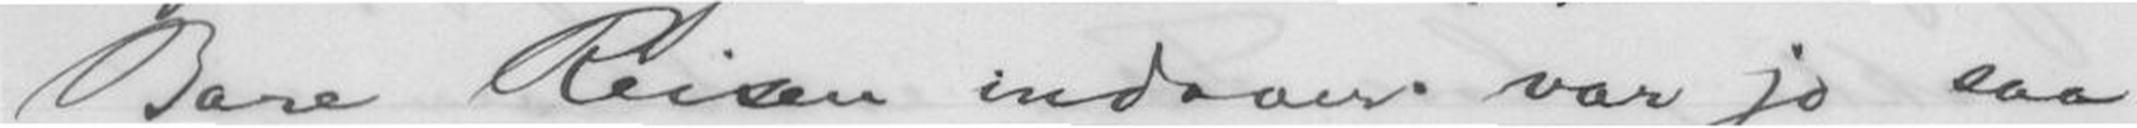Bare Reisen indover var jo saa
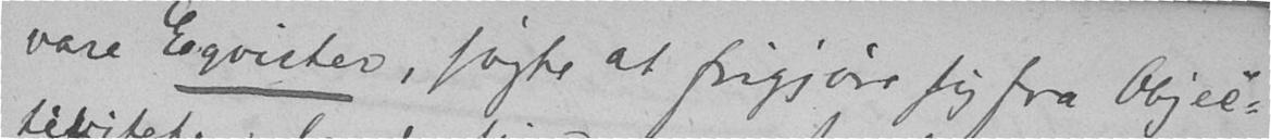vare Egoister, søgte at frigjøre sig fra Objec-
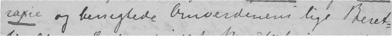raxie og benegtede Omverdenens lige Beret-
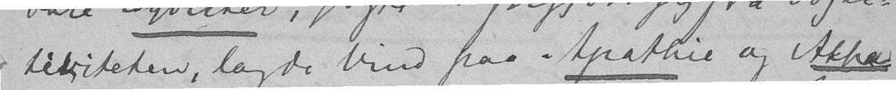tiviteten, lagde Vind paa Apathie og Atha-
